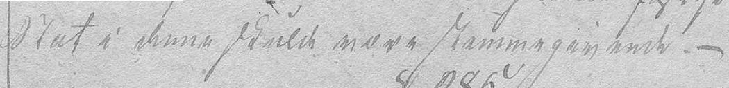Stat i denne skulde være stemmegivende. –
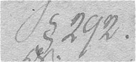§ 292.
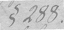§ 288.
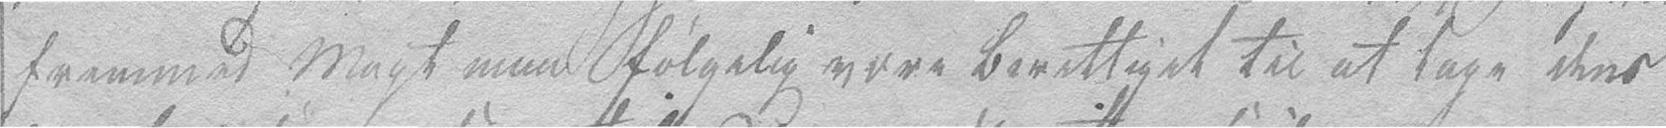fremmed Magt maa følgelig være berettiget til at tage dens
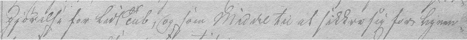gjørelse for lidt Tab, og som Middel til at sikkre sig for lignen-
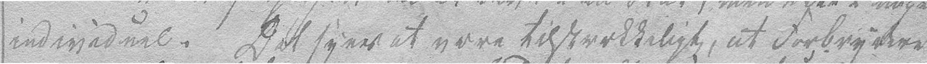individuel. Det synes at være tilstrækkeligt, at Forbrydere
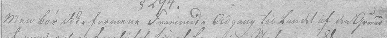Man bør ikke formene Fremmede Adgang til Landet af den Grund
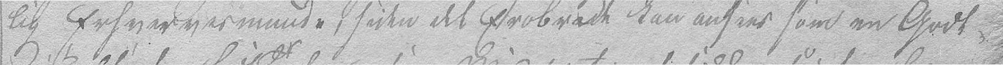lig Erhvervelsesmaade, siden det Erobrede kan ansees som en Godt-
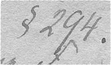§ 294.
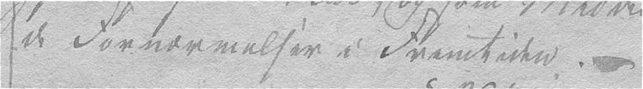de Fornærmelser i Fremtiden. –
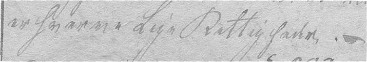erhverve lige Rettigheder. –
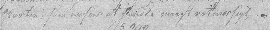Partie, som ansees at handle meest retmæssigt. –
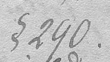§ 290.
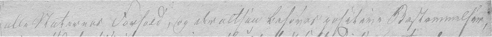alle Staternes Forhold, og der altsaa behøves positive Bestemmelser,
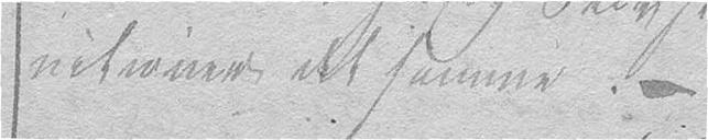nitioner det samme. –
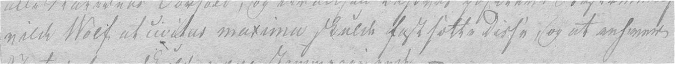vilde Wolf at civitas maxima skulde fastsætte disse, og at enhver
